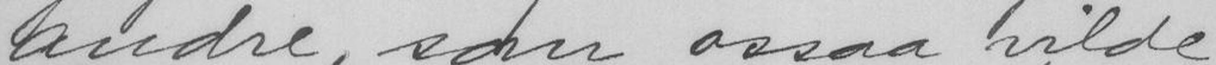andre, som ossaa vilde
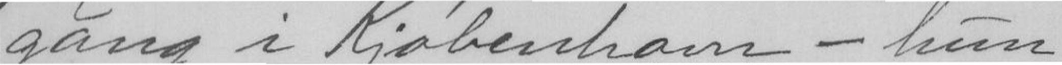gang i Kjøbenhavn - hun
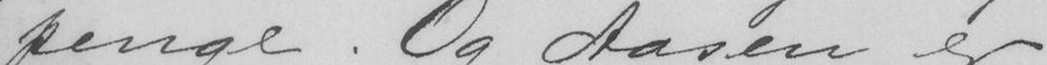penge. Og Aasen er
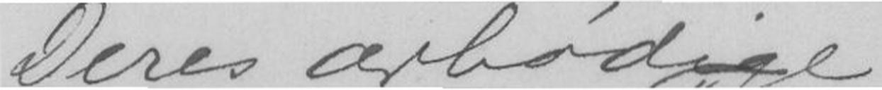Deres ærbødige
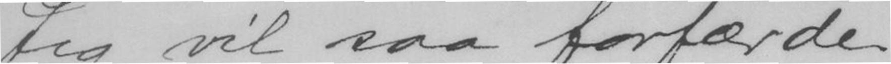Jeg vil saa forfærde-
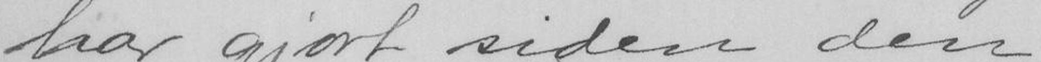har gjort siden den
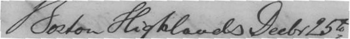Boston Highlands Decbr 25te
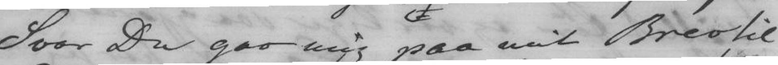Svar Du gav mig paa mit Brev til
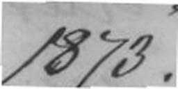1873.
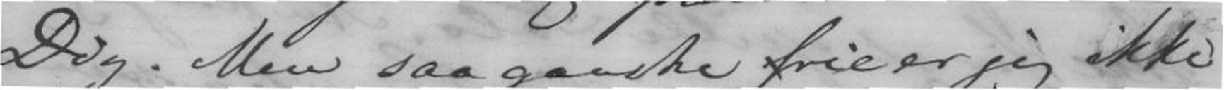Dig. Men saa ganske frie er jeg ikke
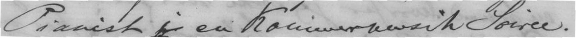Pianist i en Kammermusik Soiree.
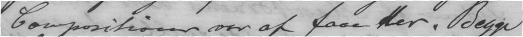Compotioner var af faae her. Begge
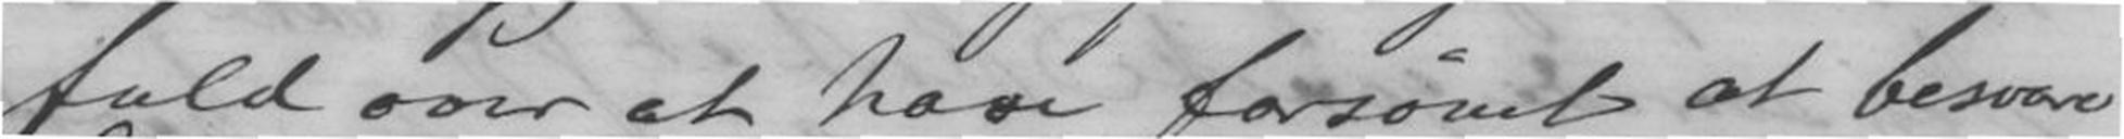fuld over at have forsømt at besvare
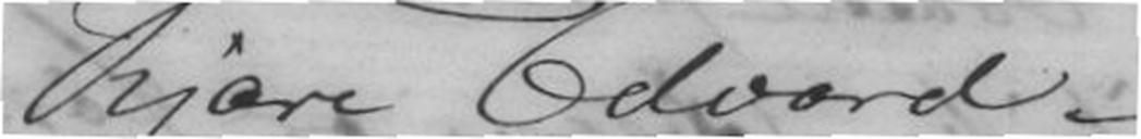Kjære Edvard.
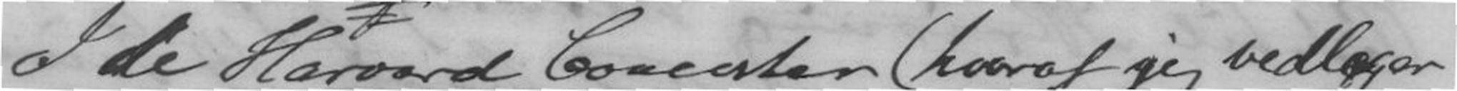I de Harvord Concerter (hvoraf jeg vedleger
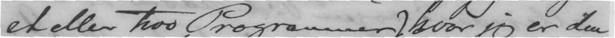et eller two Programmer) hvor jeg er den
